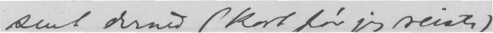sent derned (kort før jeg reiste)
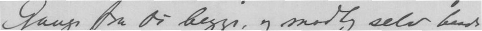Gange fra os begge, og modtag selv baade
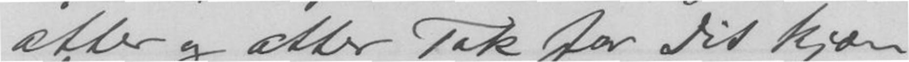atter og atter Tak for Dit kjære
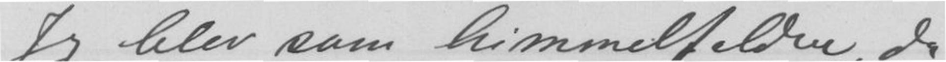Jeg blev saa himmelfalden, da
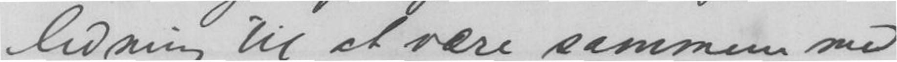ledning til at være sammen med
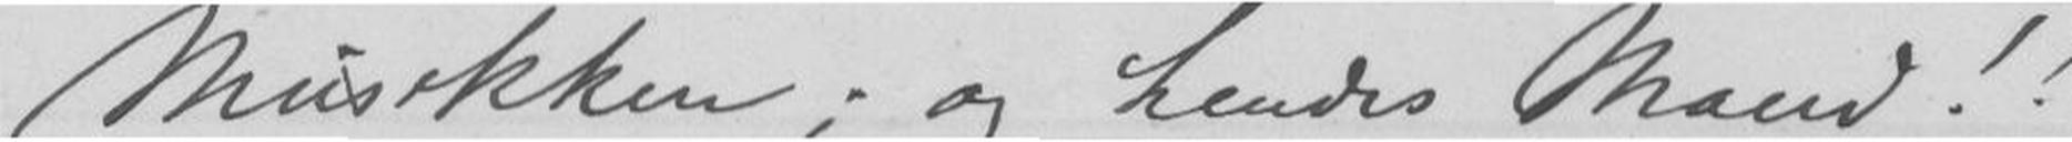Musikken; og hendes Mand!!
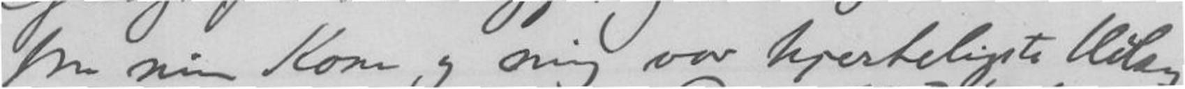fra min Kone og mig vor hjerteligste Hilsen
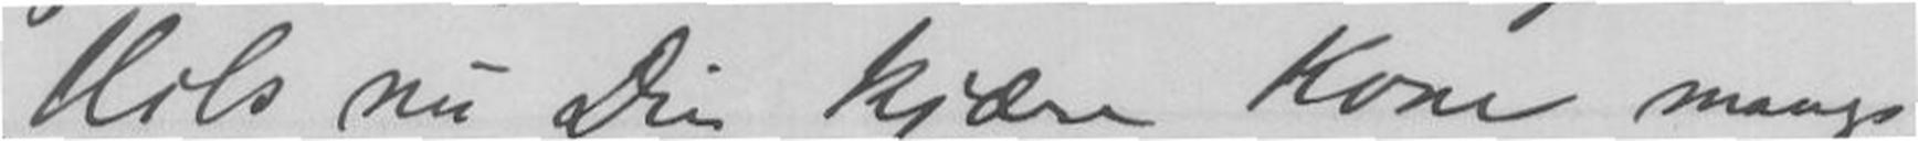Hils nu Din Kone mange
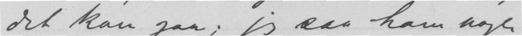det kan gaa; jeg saa ham nogle
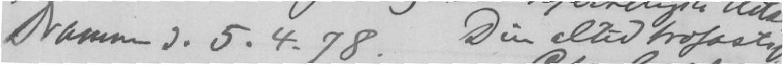Drammen d. 5.4.78 Din altid trofaste Ven
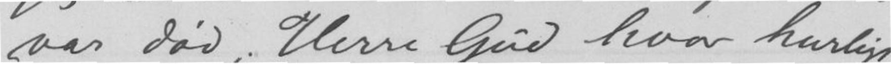var død, Herre Gud hvor hurtigt
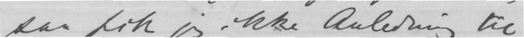saa fik jeg ikke Anledning til
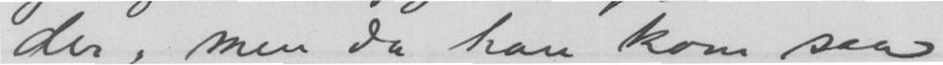der, men da han kom saa
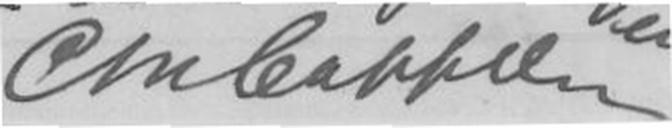Chr. Cappelen
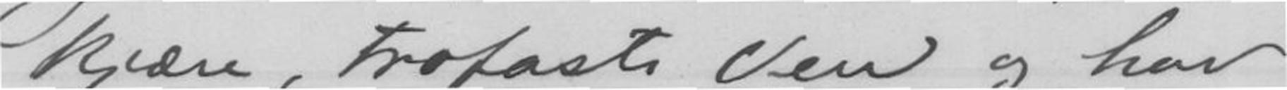kjære, trofaste Ven og hav
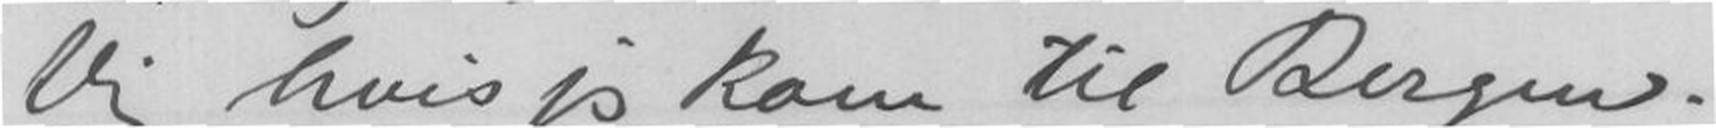Dig hvis jeg kom til Bergen.
